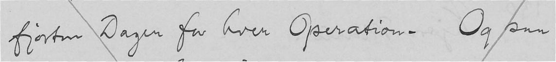fjorten Dager for hver Operation. Og saa
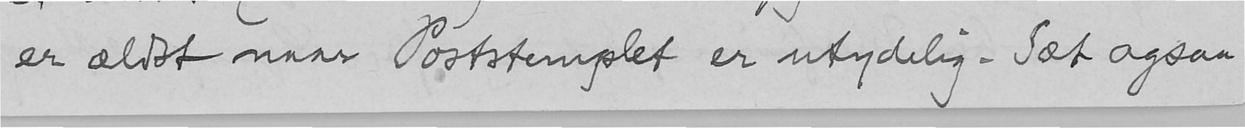er ældst naar Poststemplet er utydelig. Sæt ogsaa
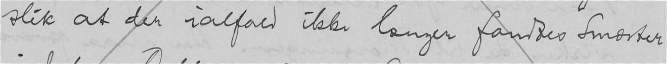slik at der ialfald ikke længer fandtes Smærter
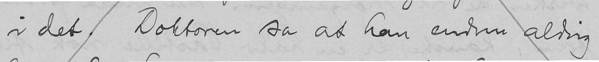i det. Doktoren sa at han endnu aldrig
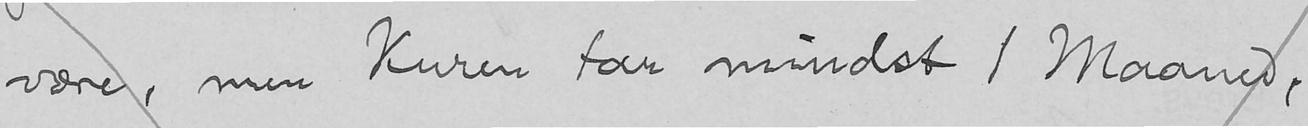være, men Kuren for mindst 1 Maaned,
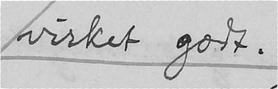virket godt.
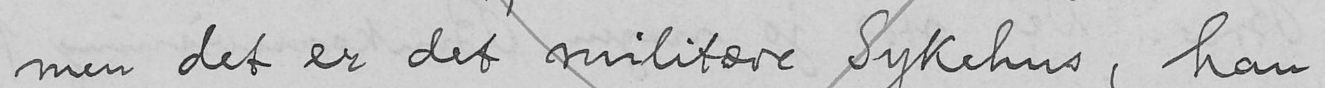men det er det militære Sykehus, han
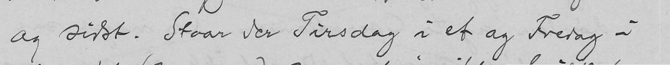og sidst. Staar der Tirsdag i et og Fredag i
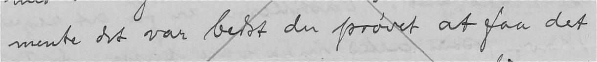mente det var bedst du prøvet at faa det
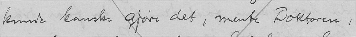kunde kanske gjøre det, mente Doktoren,
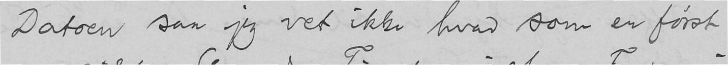Datoen saa jeg vet ikke hvad som er først
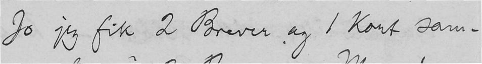Jo jeg fik 2 Brever og 1 Kort sam-
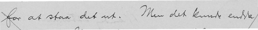for at staa det ut. Men det kunde endda
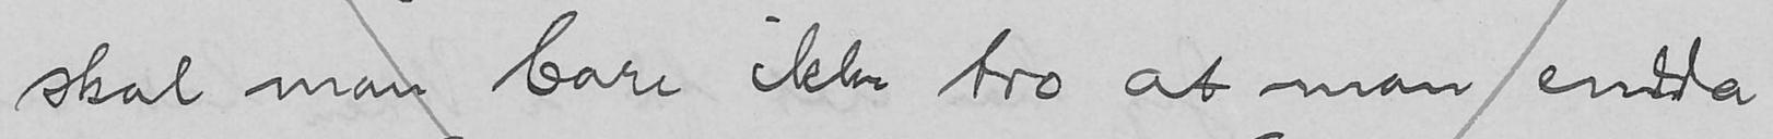skal man bare ikke tro at man endda
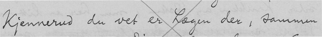Kjennerud du vet er Lægen der, sammen
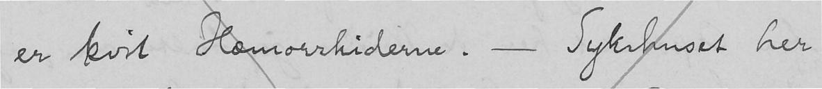er kvit Hæmorrhiderne. - Sykehuset her
